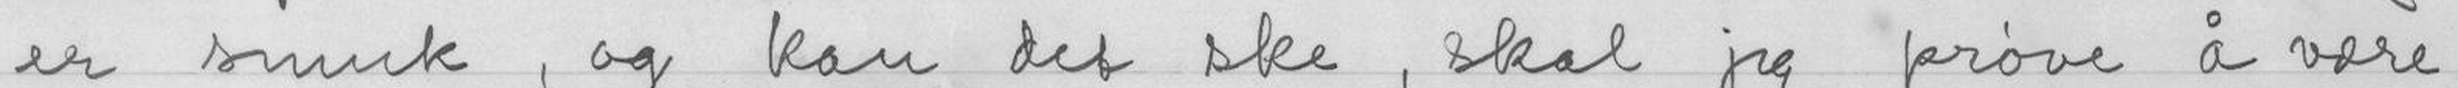er smuk, og kan det ske, skal jeg prøve å være
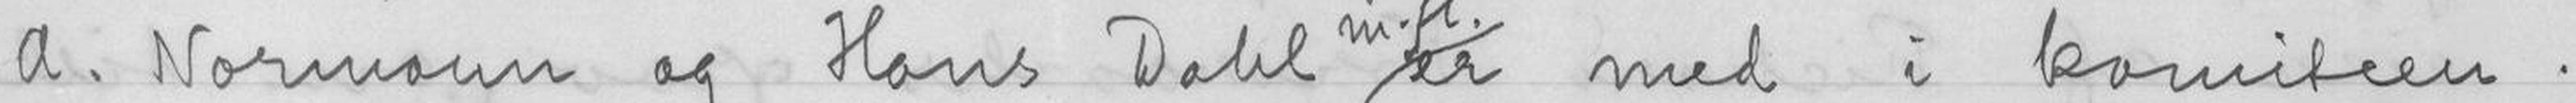A. Normannn og Hans Dahl er med i komiteen.
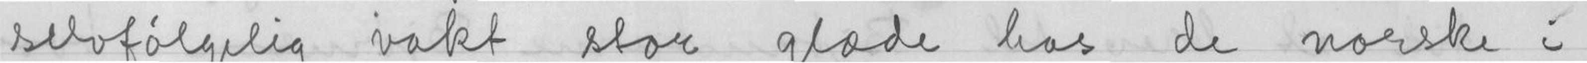selvfølgelig vakt stor glæde hos de norske i
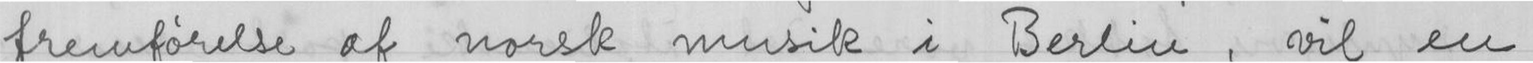fremførelse af norsk musik i Berlin, vil en
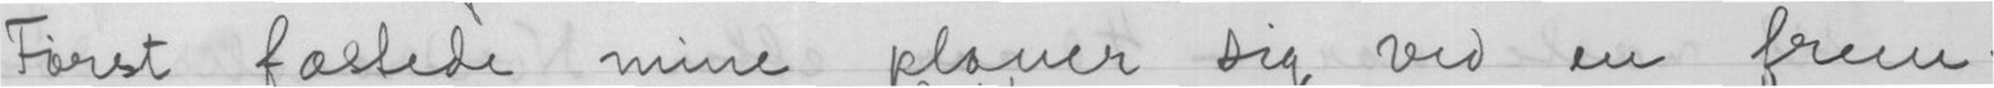Først fæstede mine planer sig ved en frem-
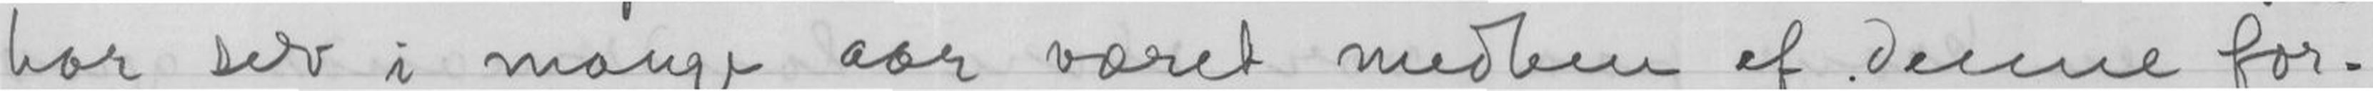har selv i mange aar været medlem af denne for-
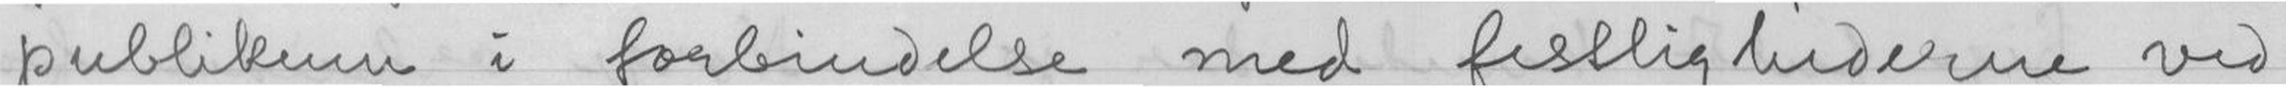publikum i forbindelse med festlighederne ved
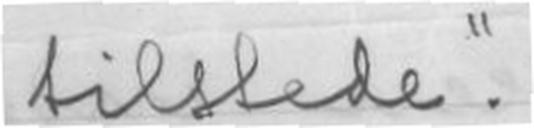tilstede".
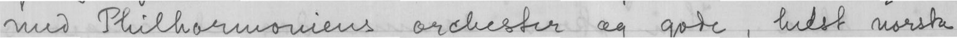med Philharmoniens orchester og gode, helst norske
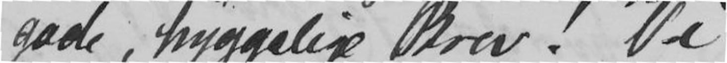gode, hyggelige Brev! Vi
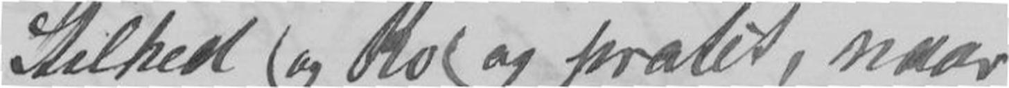Stilhed og Ro og pratet, naar
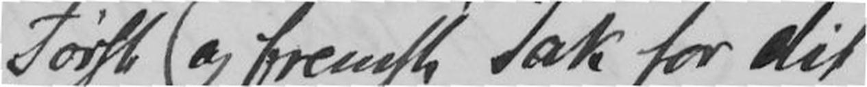Først og fremst Tak for dit
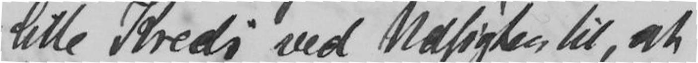lille Kreds ved Udsigten til, at
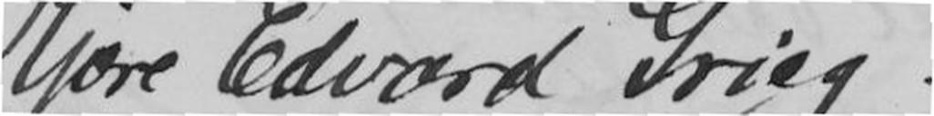Kjære Edvard Grieg!
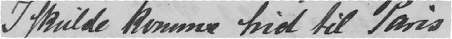I skulde komme hid til Paris
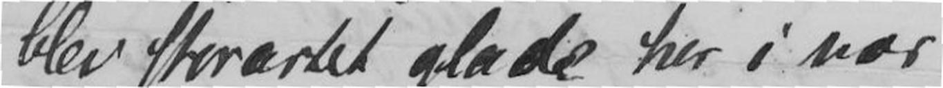blev storartet glade her i vor
